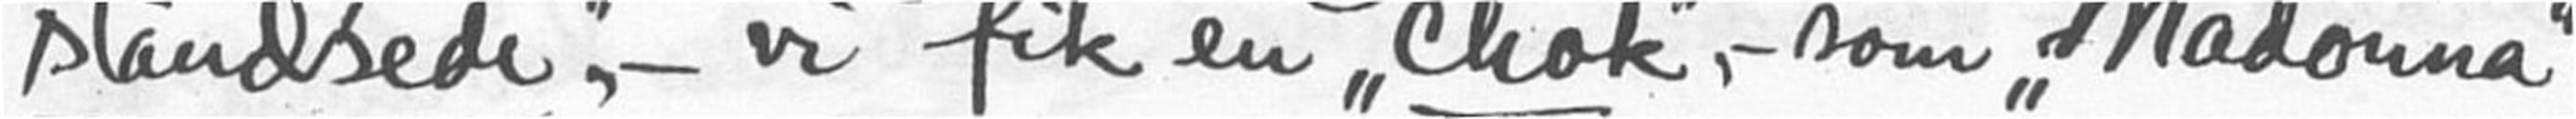standsede", - vi fik en "chok", - som "Madonna"
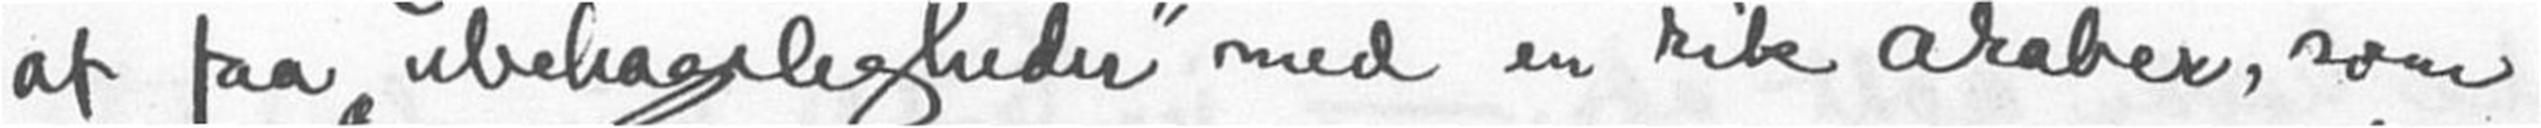at faa "ubehageligheder" med en rik araber, som
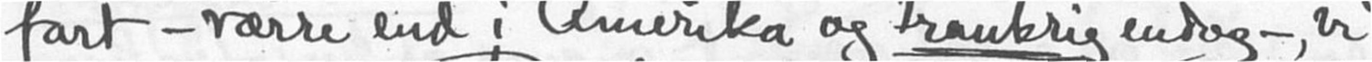fart - værre end i Amerika og Frankrig endog -, vi
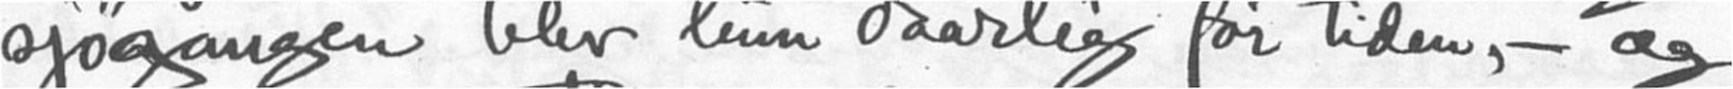sjøgangen blev hun daarlig for tiden, - og
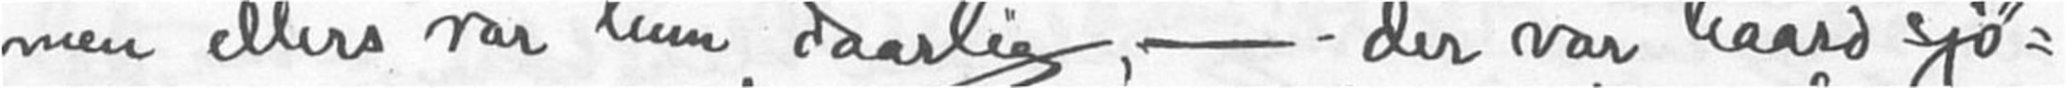men ellers var hun daarlig, - der var haard sjø-
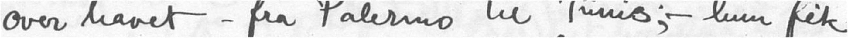over havet - fra Palermo til Tunis;- hun fik
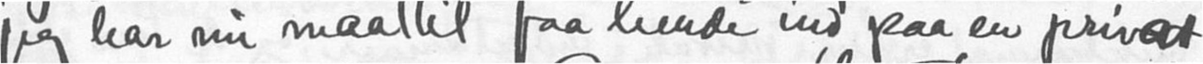jeg har nu maatet faa hende ind paa en privat
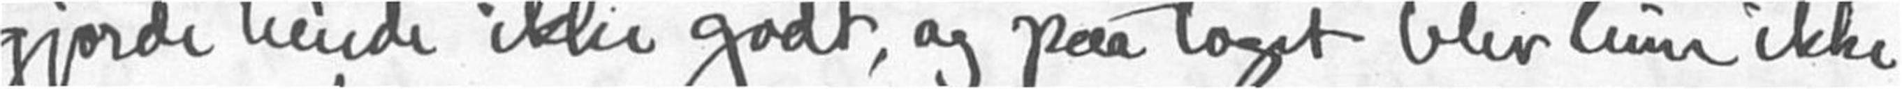gjorde hende ikke godt, og paa toget blev hun ikke
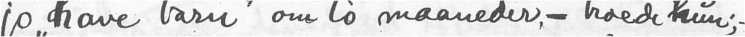jo "have barn" om to maaneder, troede hun;
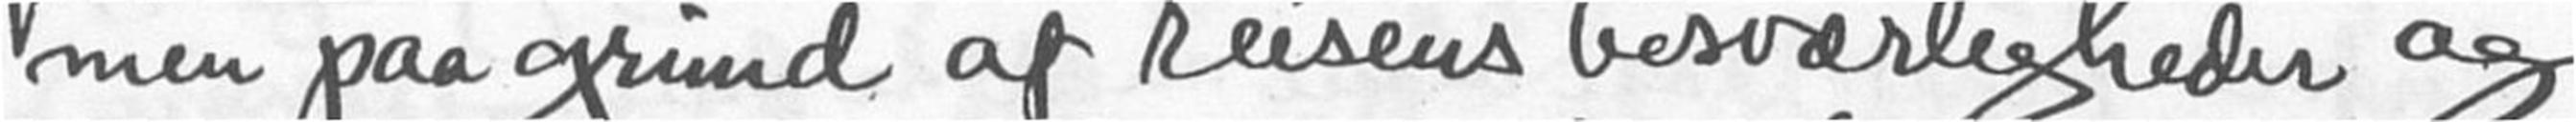men paa grund af reisens besværligheder og
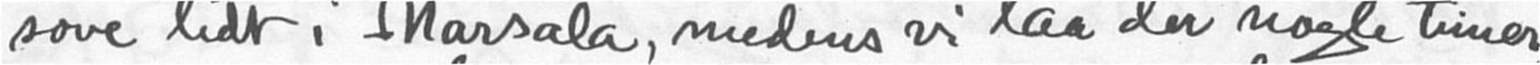sove lidt i Marsala, medens vi laa der nogle timer,
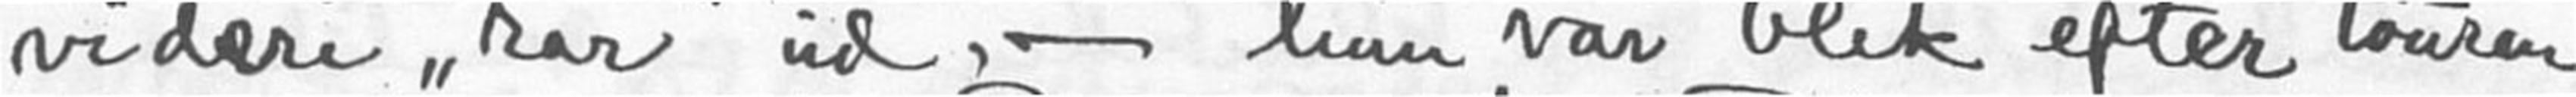videre "rar" ud, - hun var blek efter touren
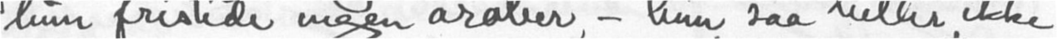hun fristede ingen araber - hun saa heller ikke
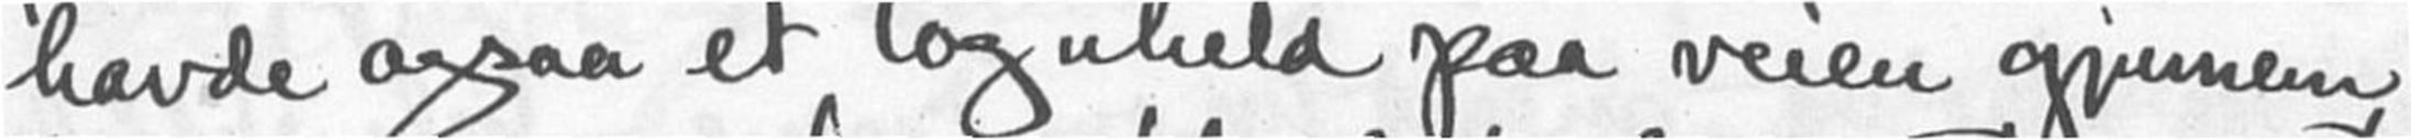havde ogsaa et toguheld paa veien gjennem
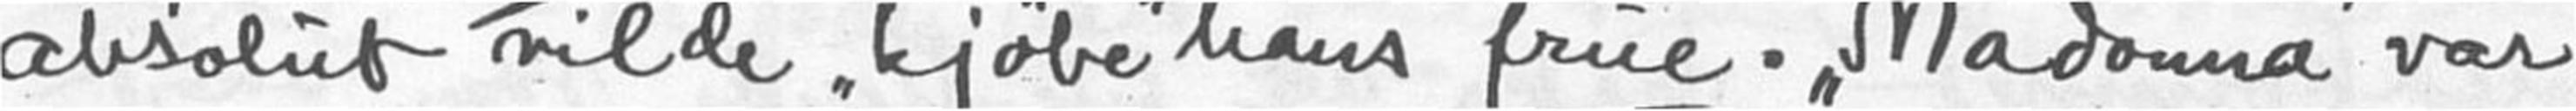absolut vilde "kjøbe" hans frue. "Madonna" var
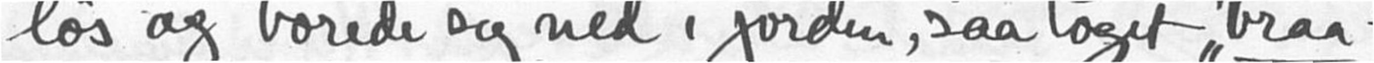løs og borede sig ned i jorden, saa toget "braa-
